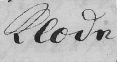Klæde
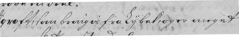groft, som bringis fra Lybek, og er meget
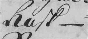Rask
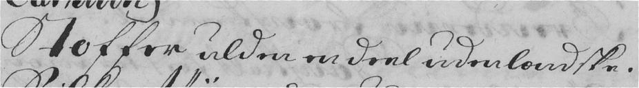Stoffer ulden en deel udenlændske.
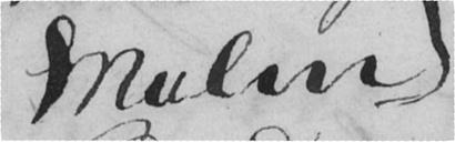Malm
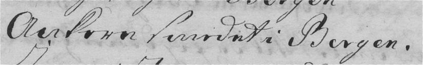Ankere smedet i Bergen.
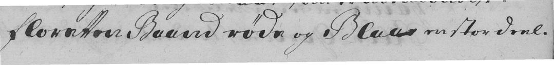Floretten Baand røde og Blaa en stor deel.
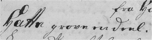Hatte grove en deel.
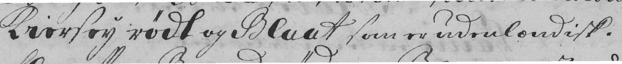Kiersey rødt og Blaat som er udenlændisk.
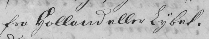fra Holland eller Lybek.
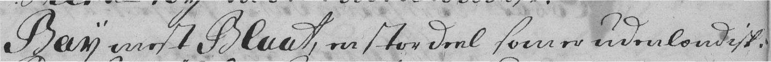Bay mest Blaat, en stor deel som er udenlændisk.
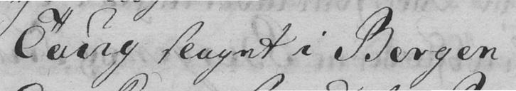Taug slaget i Bergen
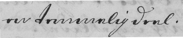en temmelig deel.
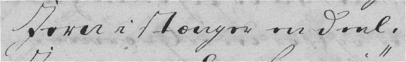Iern i stænger en deel.
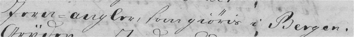Iern-angler, som giøris i Bergen.
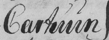Cartuun
